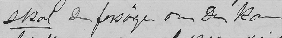skal Du forsøge om Du kan
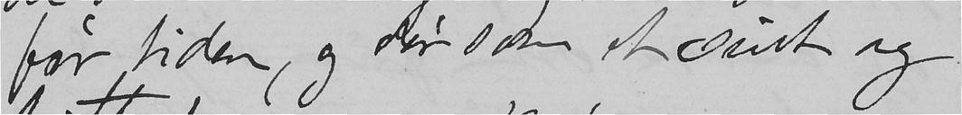før tiden, og der som et sint og
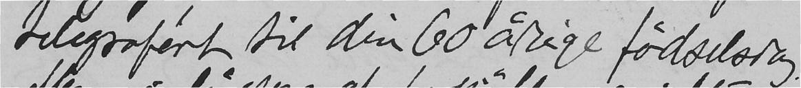telegrafert til din 60 årige fødselsdag.
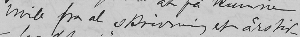hvile fra al skrivning et års tid -
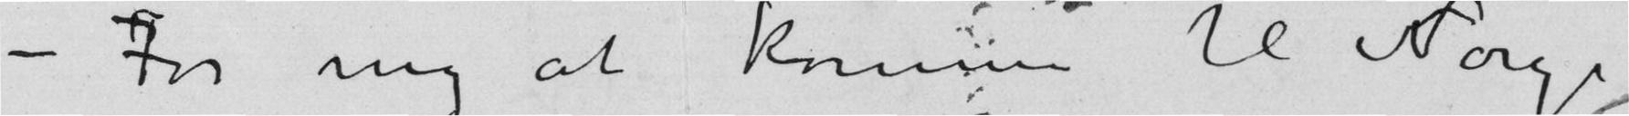– For mig at komme til Norge
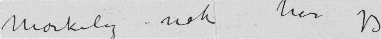Mærkelig nok har jeg
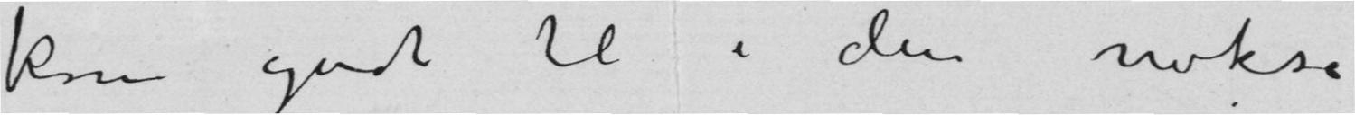kom godt til i denne nokså
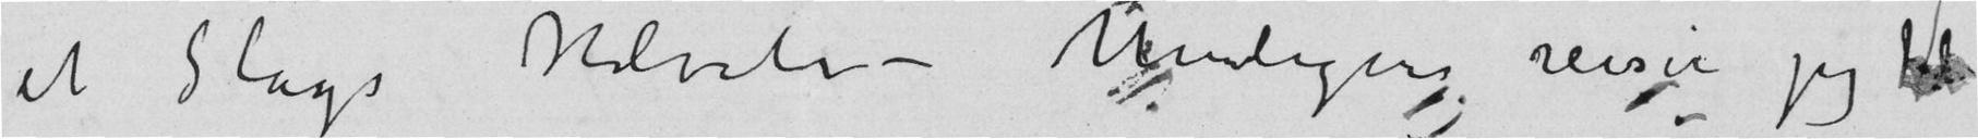et Slags Helvede – Rimeligvis reiser jeg til
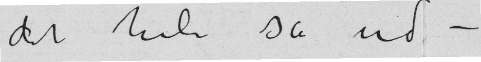det hele så ud –
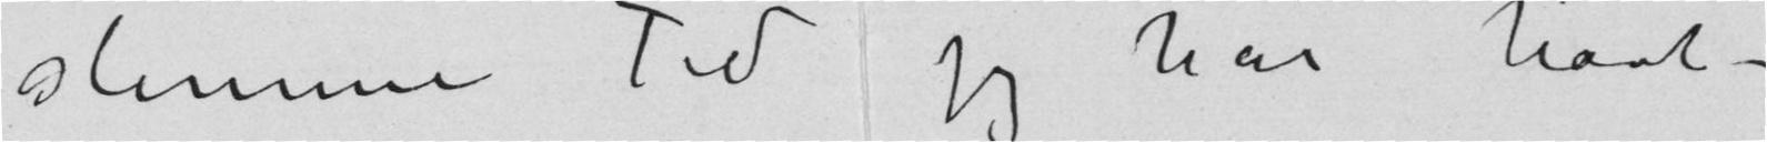slemme Tid jeg har havt –
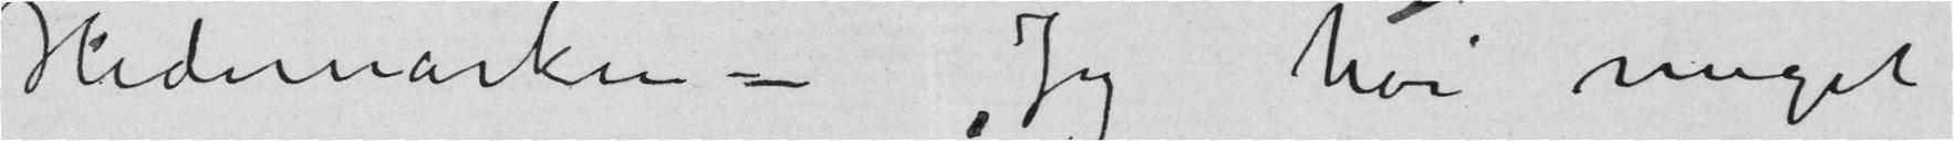Hedemarken – Jeg har meget
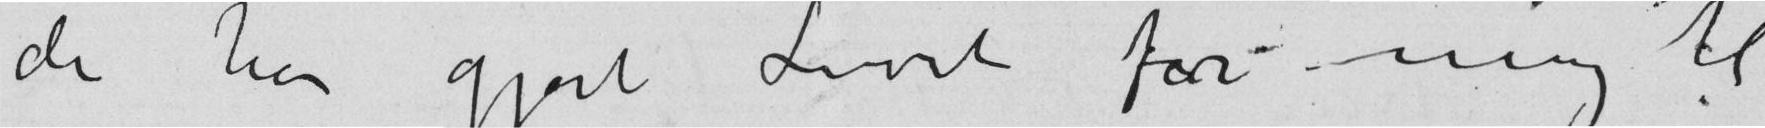der har gjort Livet for mig til
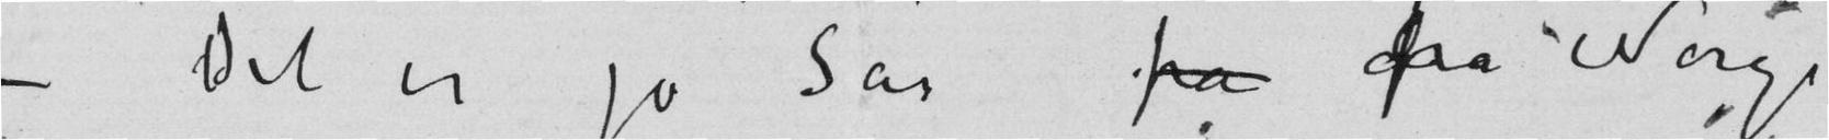– Det er jo Sårfra Norge
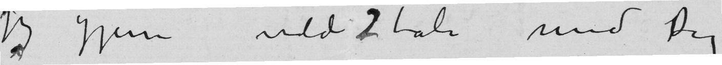jeg gjerne vildetale med Dig
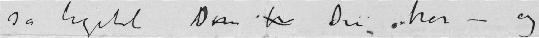så ligetil som Du tror – og
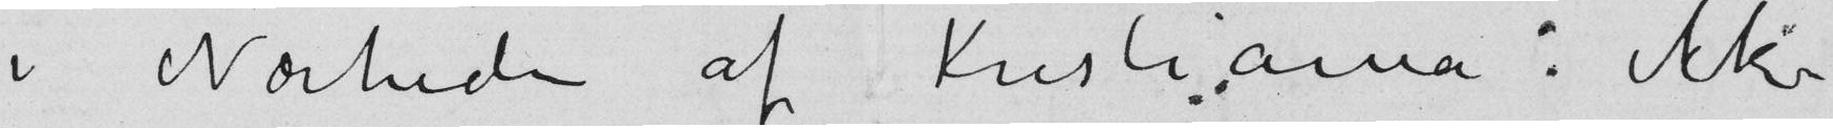i nærheten av Kristiania: ikke
